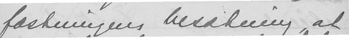fæstningens besætning, at
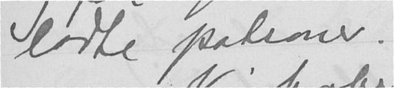ladte patroner.
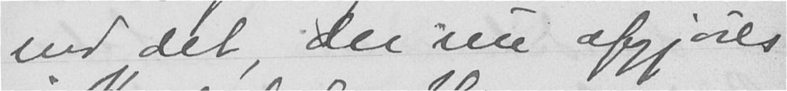end det, der nu afgjøres
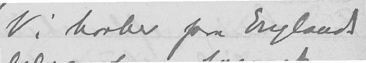Vi haaber paa Englands
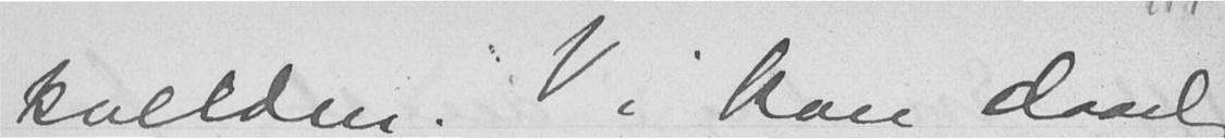kvelden. Vi kan daarlig
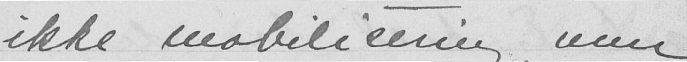ikke mobilisering, men
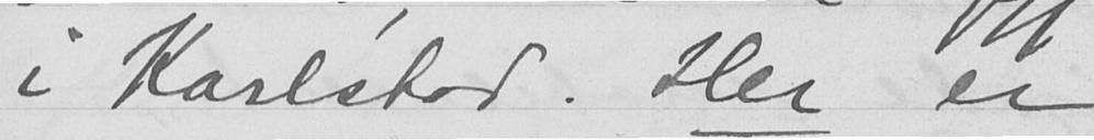i Karlstad. Her er
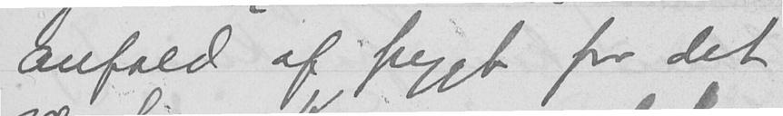anfald af frygt for det
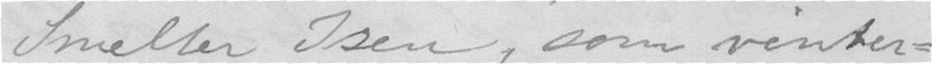Smelter Isen, som vinter-
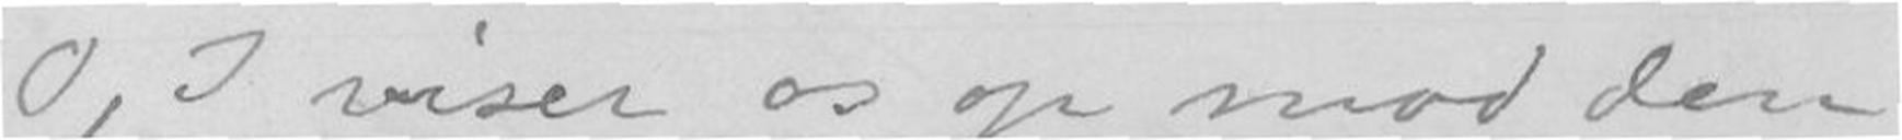O, I viser os op mod den
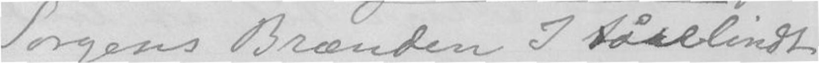Sorgens Brænden I tårelindt
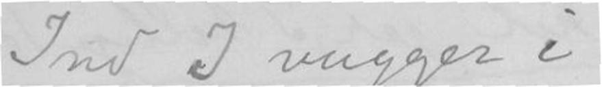Ind I vugger i Sje Sindet
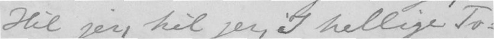Hil jer, hil jer, I hellig To-
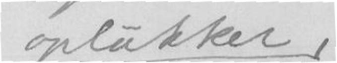oplukker,
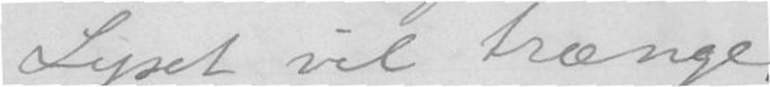Lyset vil trænge.
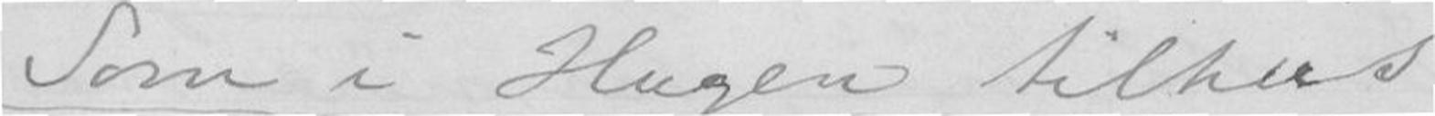Som i Hugen tilhus
